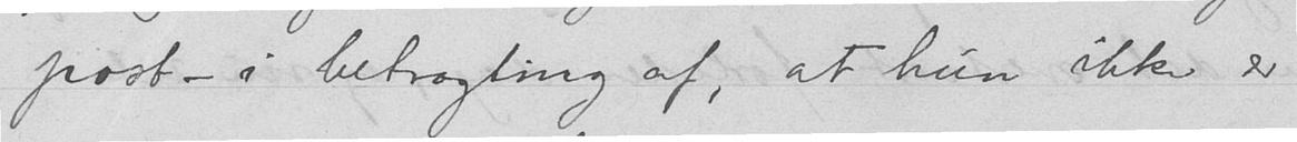post – i betragting af, at hun ikke er
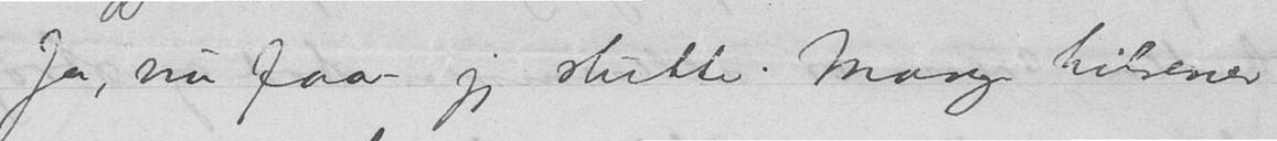Ja, nu faar jeg slutte. Mange hilsener
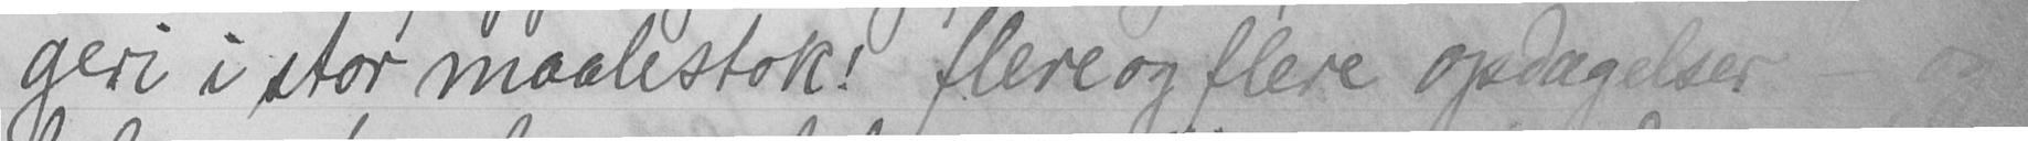geri i stor maalestok! flere og flere opdagelser - og
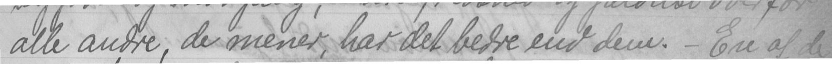alle andre, de mener, har det bedre end dem. - En af de
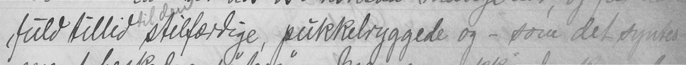fuld tillid til den stilfærdige, pukkelryggede og - som det syntes
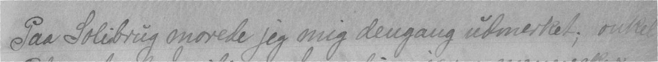Paa Solibrug morede jeg mig dengang udmerket; onkel
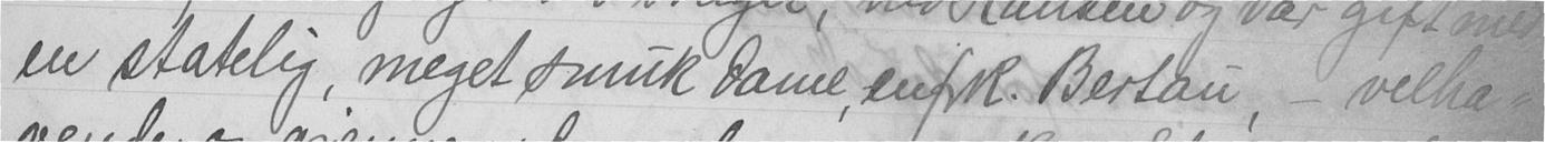en statelig, meget smuk dame, en frk. Bertan, - velha-
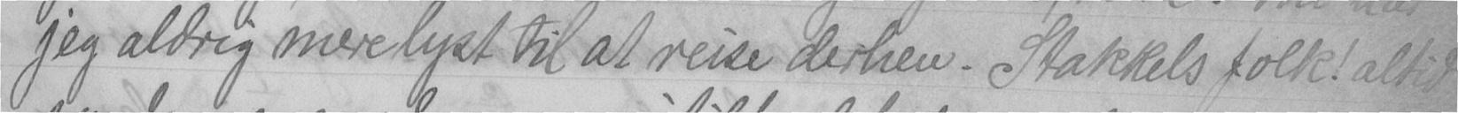jeg aldrig mere lyst til at reise derhen. Stakkels folk! altid
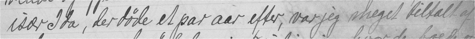især Ida, der døde et par aar efter, var jeg meget tiltalt af
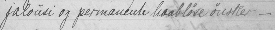jalousi og permanente haabløse ønsker -
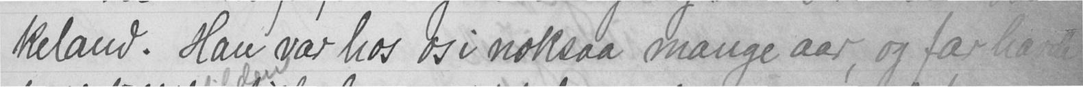keland. Han var hos os i noksaa mange aar, og far havde
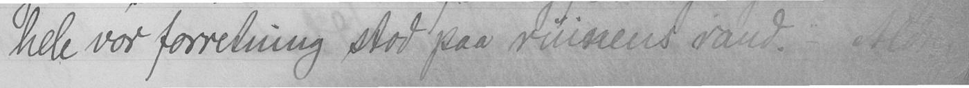hele vor forretning stod paa ruinens rand. - Aldrig
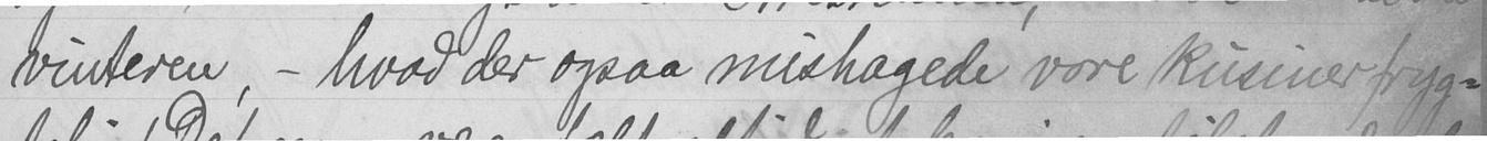vinteren, - hvad der ogsaa mishagede vore kusiner fryg-
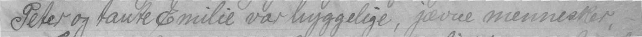Peter og tante Emilie var hyggelige, jævne mennesker, -
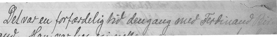Det var en forfærdelig tid dengang med Ferdinand Bir-
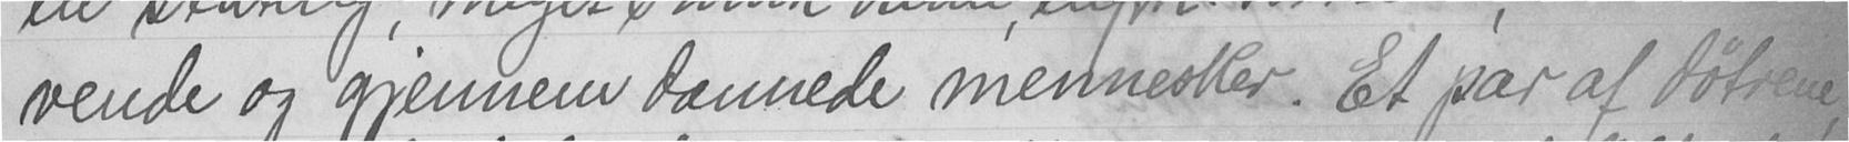vende og gjennem dannede mennesker. Et par af døtrene,
